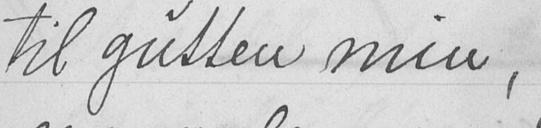til gutten min,
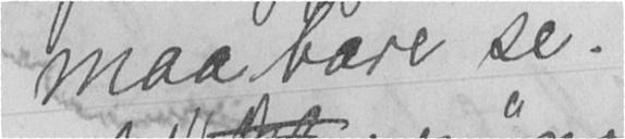maa bare se.
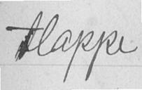tlappe
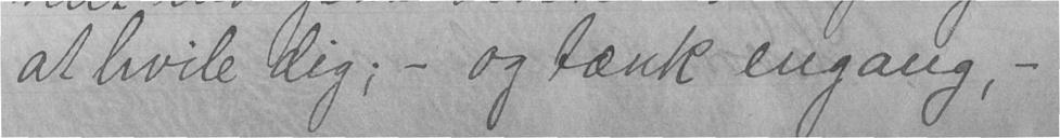at hvile dig; - og tænk engang, -
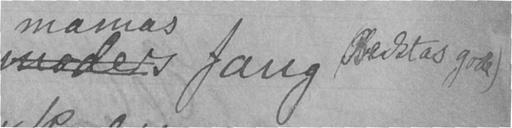moders fang (Bedstas gode)
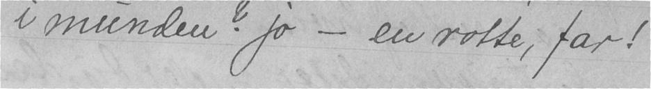i munden? Jo - en rotte, far!
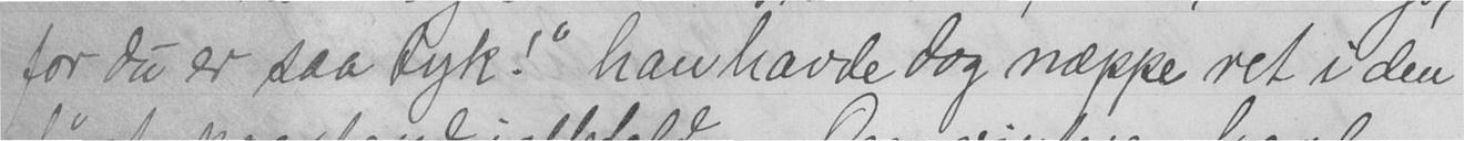for du er saa tyk!" han havde dog næppe ret i den
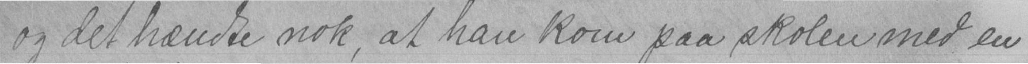og det hændte nok, at han kom paa skolen med en
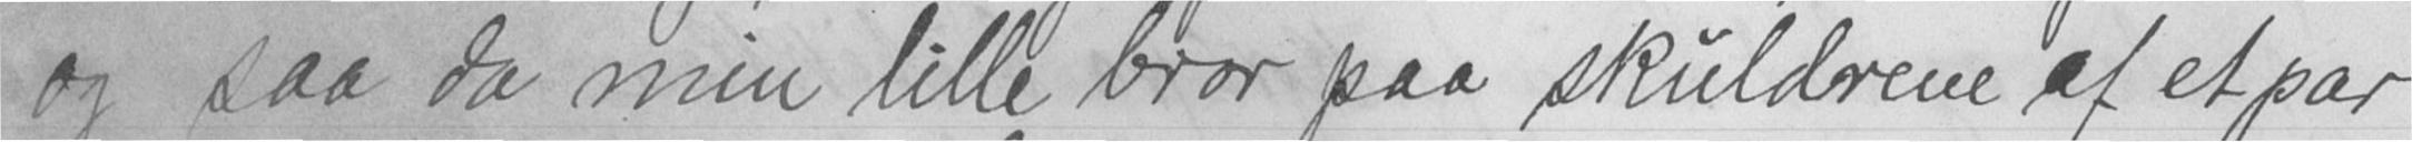og saa da min lille bror paa skuldrene af et par
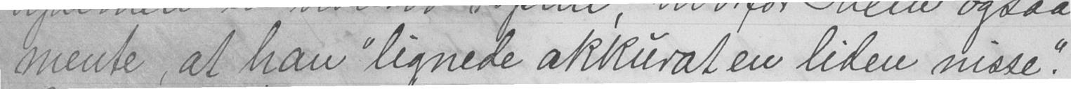mente, at han "lignede akkurat en liden nisse".
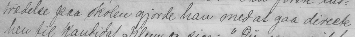trædelse paa skolen gjorde han med at gaa directe
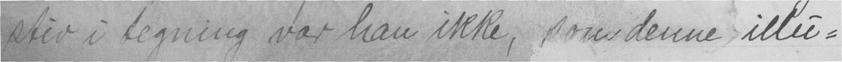stiv i tegning var han ikke, som denne illu-
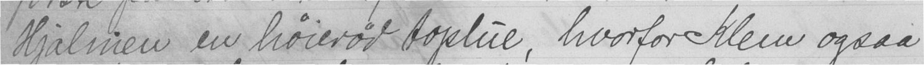Hjalmen en høierød toplue, hvorfor Klem ogsaa
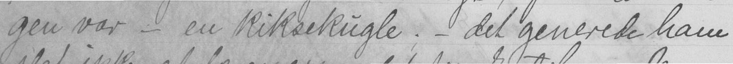gen var - en kiksekugle; - det generede ham
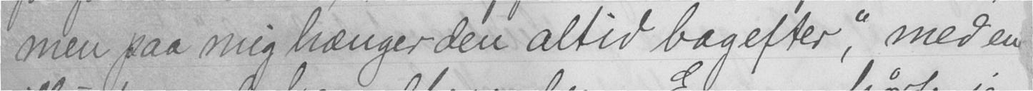men paa mig hænger den altid bagefter," med en
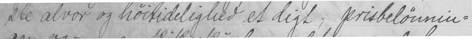ste alvor og høitidelighed et digt; prisbelønnin-
